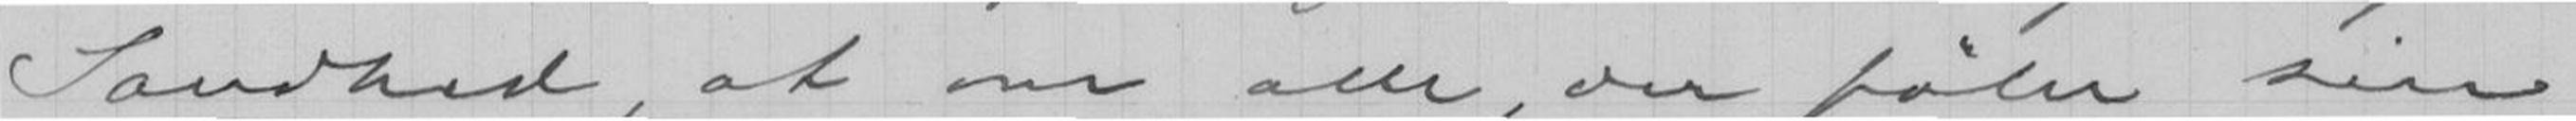Sandhed, at om alle, der føler sin
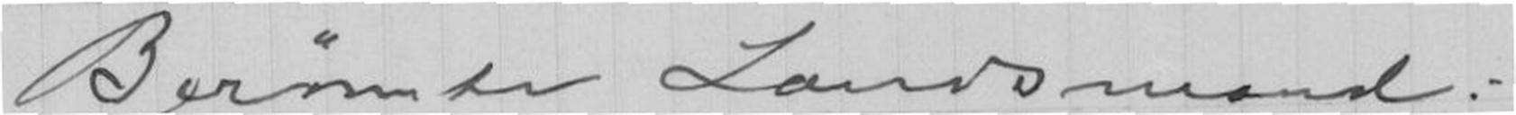Berømte Landsmand:
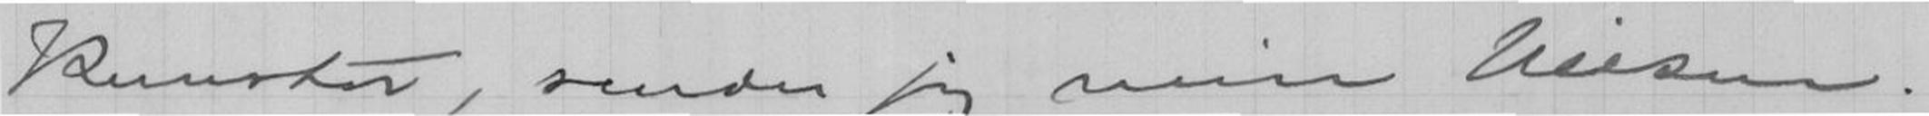kunster, sender jeg min hilsen
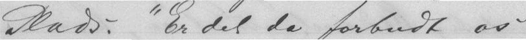Plads. "Er det da forbudt os
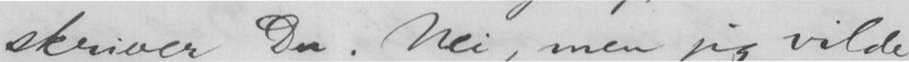skriver Du. Nei, men jeg vilde
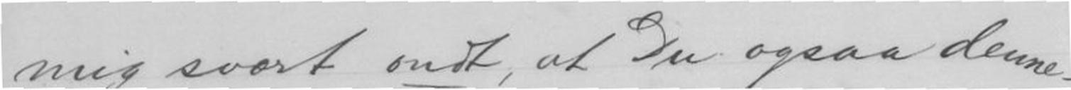mig svært ondt, at Du ogsaa denne-
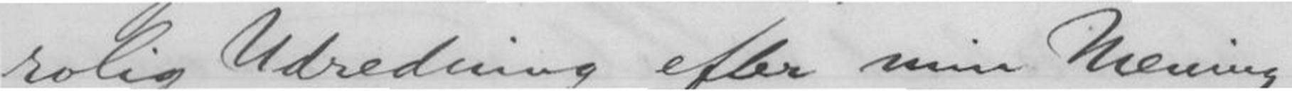rolig Udredning efter min Mening
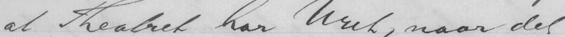at Theatret har Uret, naar det
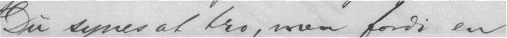Du synes at tro, men fordi en
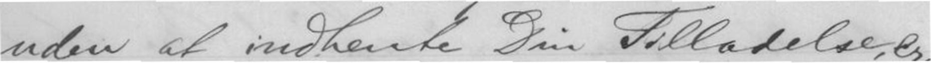uden at indhente Din Tilladelse, er
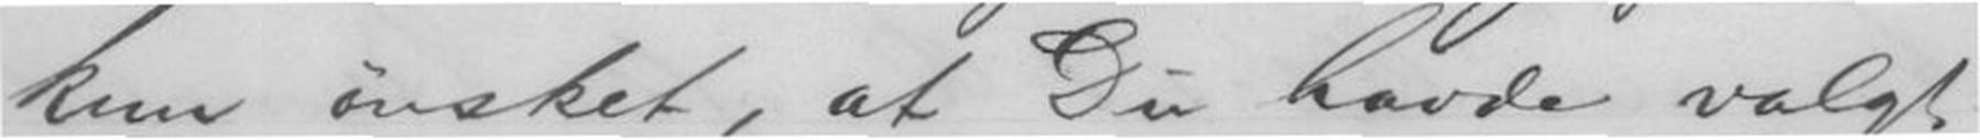kun ønsket, at Du havde valgt
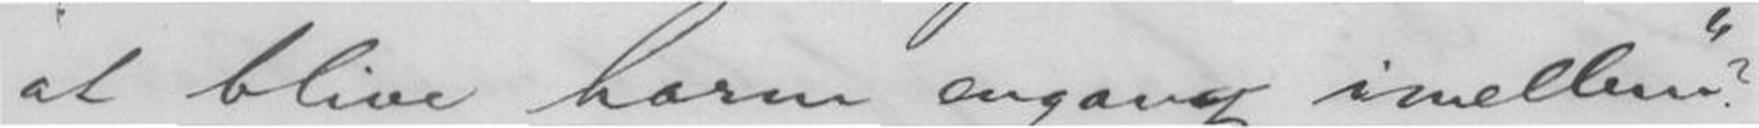at blive harm engang imellem"?
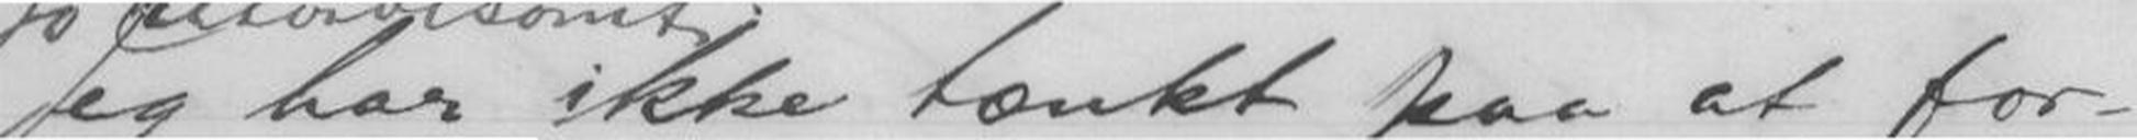Jeg har ikke tænkt paa at for-
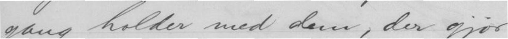gang holder med dem, der gjør
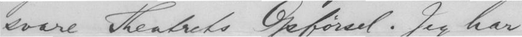svare Theatret Opførsel. Jeg har
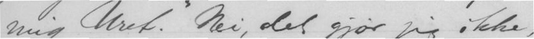mig Uret." Nei, det gjør jeg ikke,
